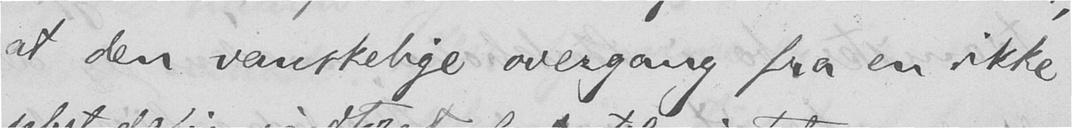at den vanskelige overgang fra en ikke
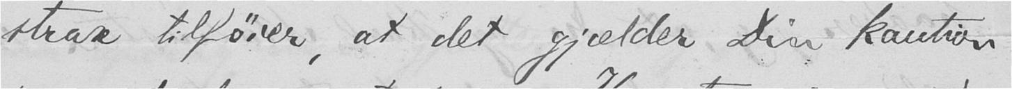strax tilføier, at det gjælder Din kaution
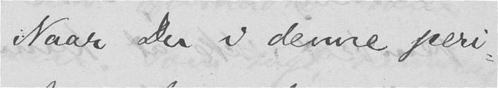Naar Du i denne peri-
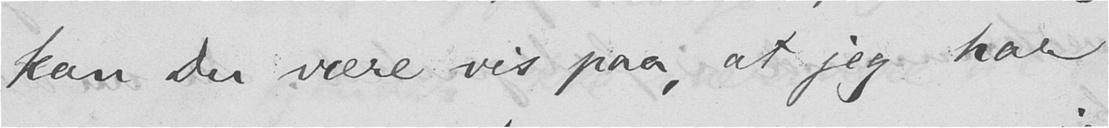kan Du være vis paa, at jeg har
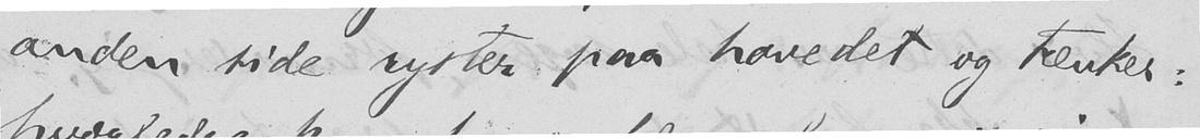anden side ryster paa hovedet og tænker:
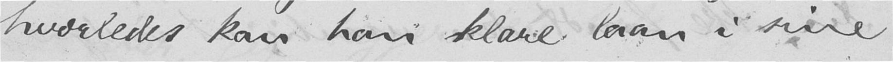hvorledes kan han klare laan i sine
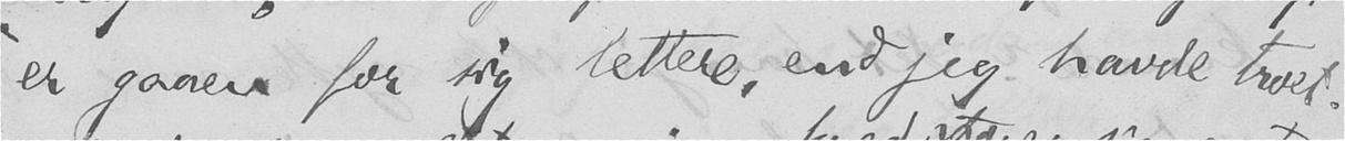er gaaen for sig lettere, end jeg havde troet.
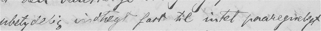ubetydelig indtægt fast til intet paaregneligt
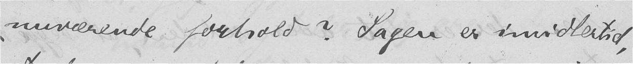nuværende forhold? Sagen er imidlertid,
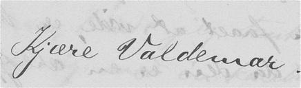Kjære Valdemar!
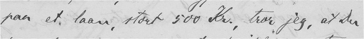paa et laan, stort 500 Kr., tror jeg, at Du
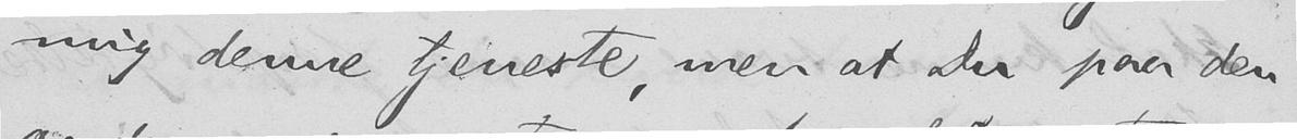mig denne tjeneste, men at Du paa den
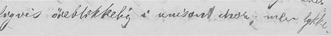ligvis øieblikkelig i unisont chor; men lykke-
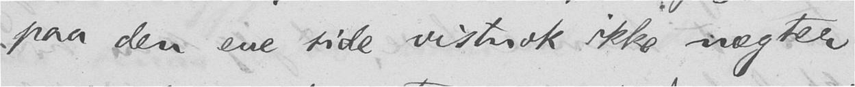paa den ene side vistnok ikke nægter
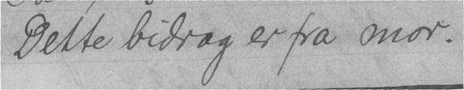Dette bidrag er fra mor.
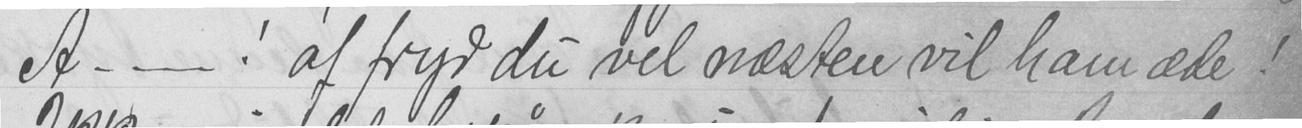A --! af fryd du vel næsten vil ham æde!
